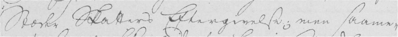Stæder Skatters Eftergivelse; men saame-
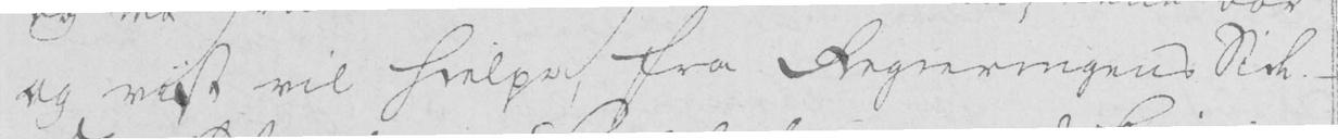og vist vil hielpe, fra Regieringens Side. -
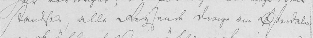standses, alle Reysende drage om Østerdalen
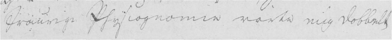traurige Physiognomie rørte mig dobbelt
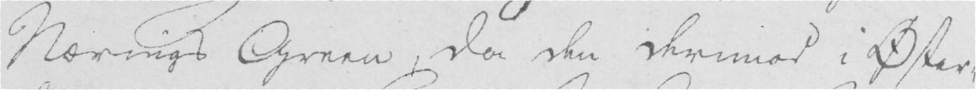Nærings Green, da den derimod i Øster-
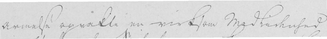armelse opvakle en virksom Modladenhed
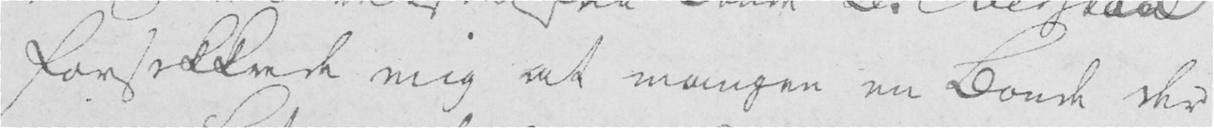forsikkrede mig at mangen en Bonde der
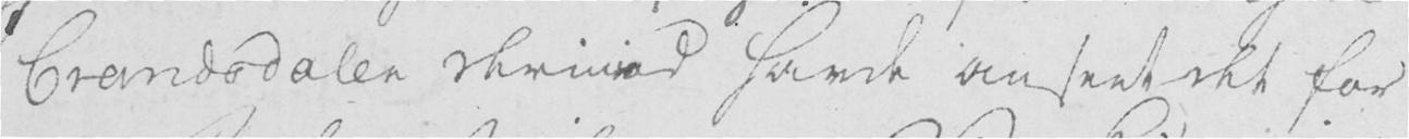brandsdalen derimod havde anseet det for
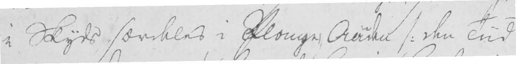i Skyds særdeles i Plange Aaden /: den Tiid
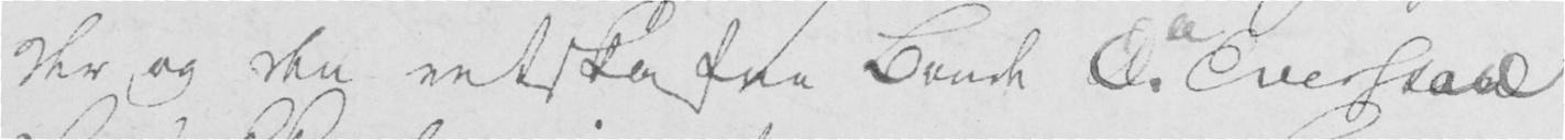der og den retskafne Bonde O. Everstad
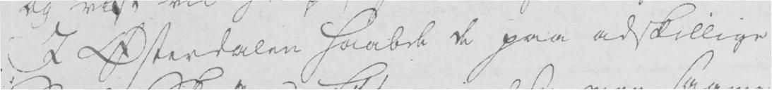I Østerdalen haabede de paa adskillige
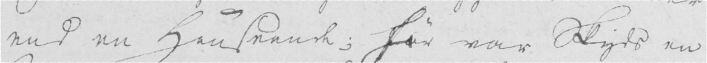end en Henseende; før var Skyld en
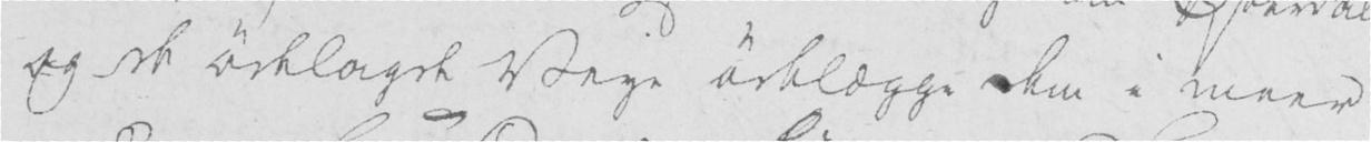og de ødelagde Veye ødelægge dem i meer
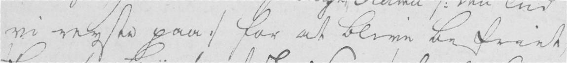vi reyste paa :/ for at blive befriet
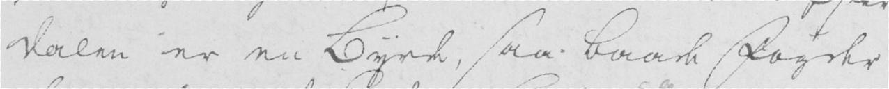dalen er en Byrde, saa baade føyede
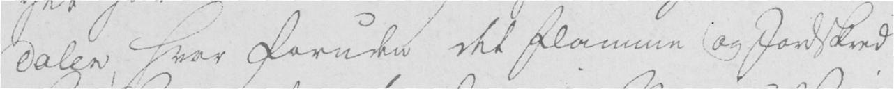dalen, hvor foruden det Flomme og Jordskred
